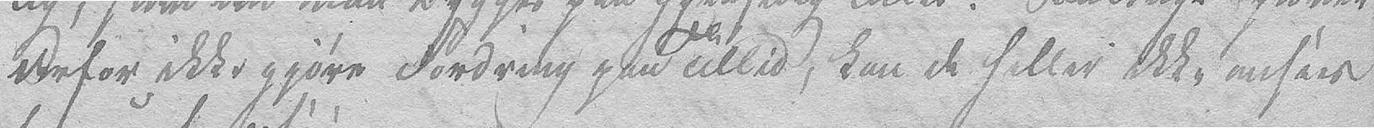derfor ikke gjøre Fordring paa Tillid, kan de heller ikke ansees
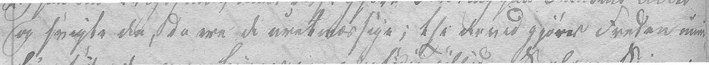og svigte den, da ere de uretmæssige; thi derved gjøres Freden umu-
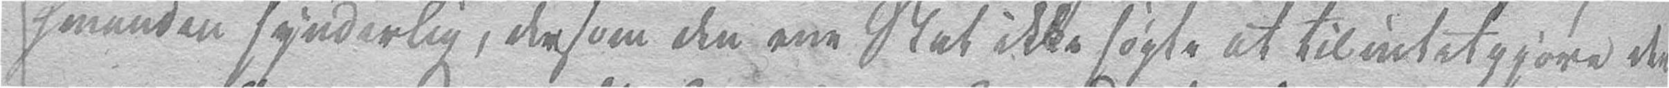hinanden synderlig, dersom den ene Stat ikke søgte at tilintetgjøre den
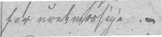for uretmæssige. –
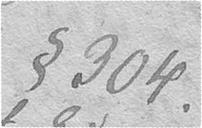§ 304.
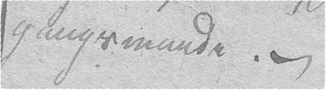gangsmaade. –
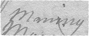Mening
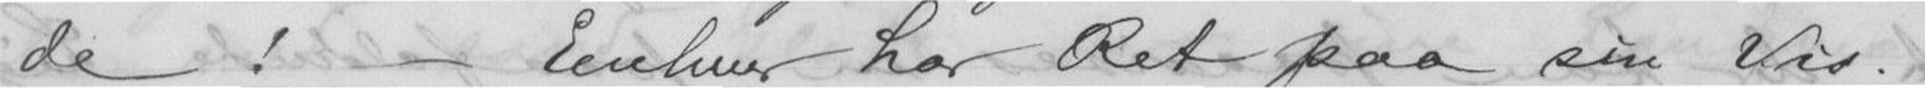de! - Enhver har Ret paa sin Vis. -
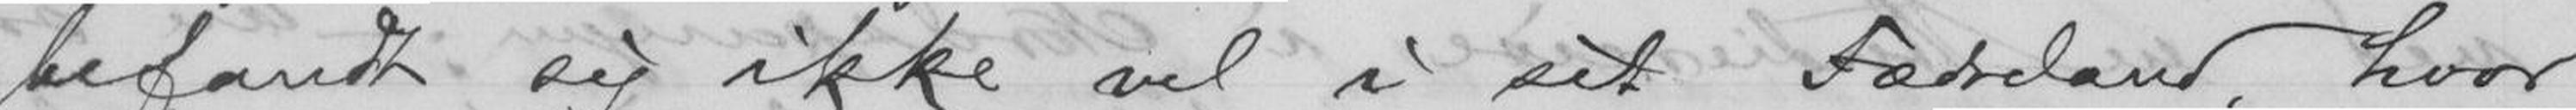befandt sig ikke vel i sit Fædreland, hvor
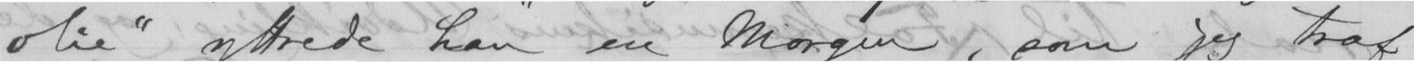olie yttrede han en Morgen, som jeg traf
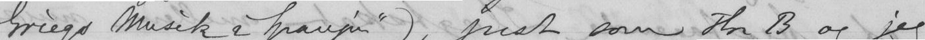Griegs Musik & Spanjen), just som stor B og jeg
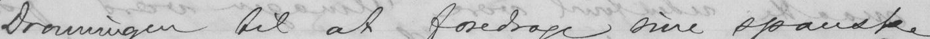Dronningen til at foredrage sine spanske
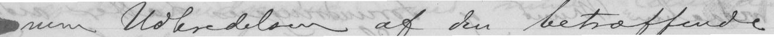gjennem Udbredelsen af den betræffende
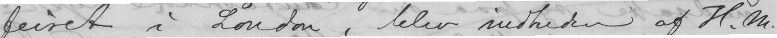feiret i London, blev indbuden af H.M.
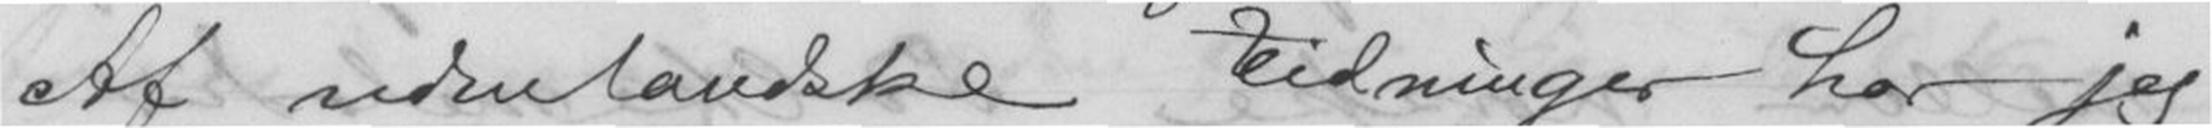Af udenlandske Tidninger har jeg
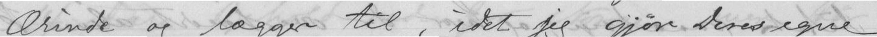Ærinde og lægger til, idet jeg gjør Deres egne
